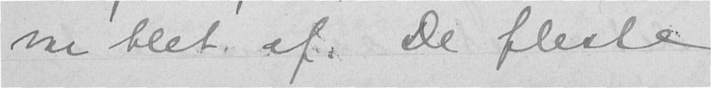var blit af. De fleste
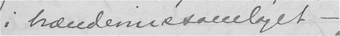i brænderissamlaget -
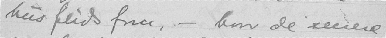husflidsforn. - hvor de senere
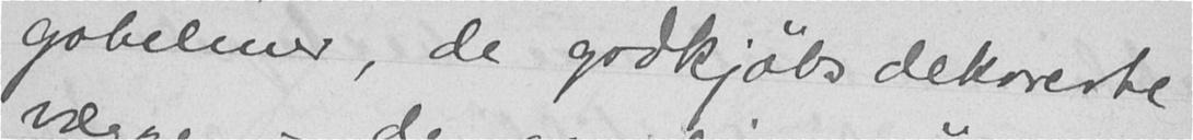gobeliner, de godkjøbs dekorerte
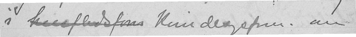i husflidsfons Kvindesagsforn. om
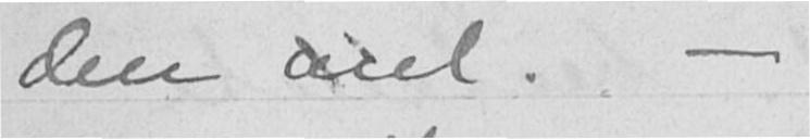den anl. -
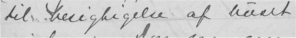til besigtigelse at huset
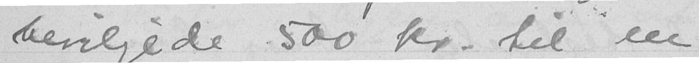bevilgede 500 kr. til en
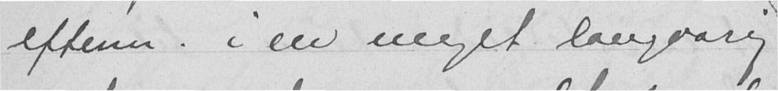efterm. i en meget langvarig
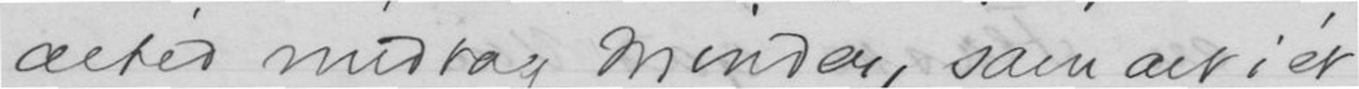altid minder, som det i et
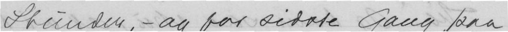under,- og for sidste Gang paa
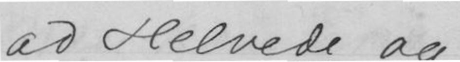ad Helvede og
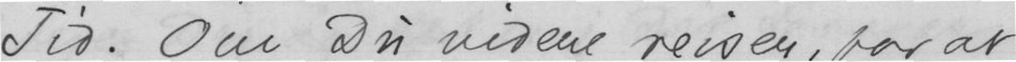Tid. Om Du videre reiser, for at
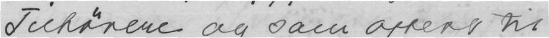Tilhørere og som oftest til
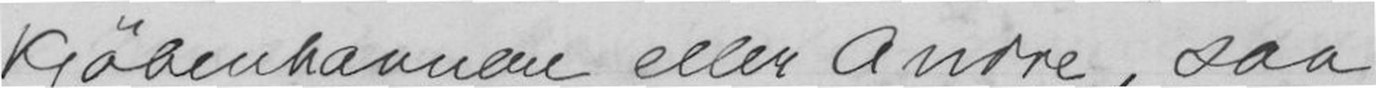Kjøbenhavnere eller Andre, saa
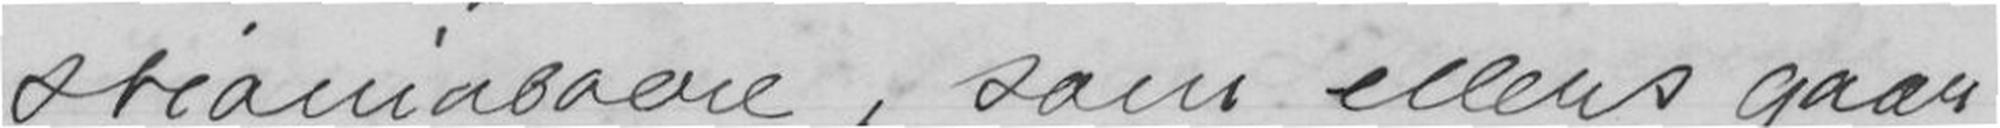stianianære, som ellers gaar
